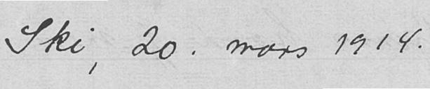Ski, 20. mars 1914.
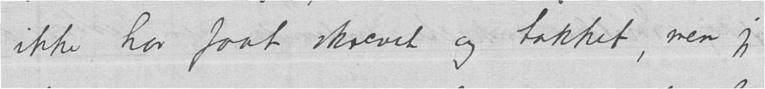ikke har faat skrevet og takket, men jeg
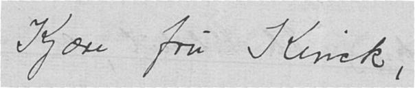Kjære fru Kinck,
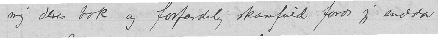mig Deres bok og forfærdelig skamfuld fordi jeg endda
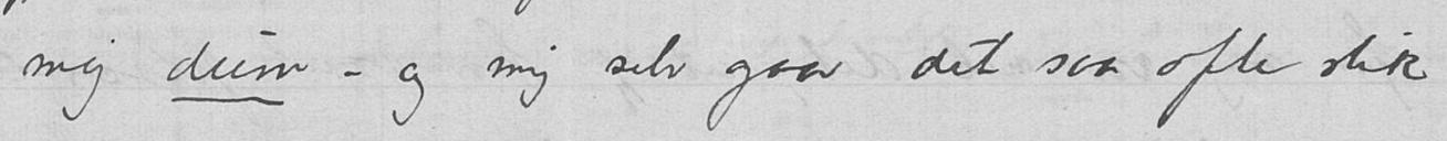mig dum – og mig selv gaar det saa ofte slik
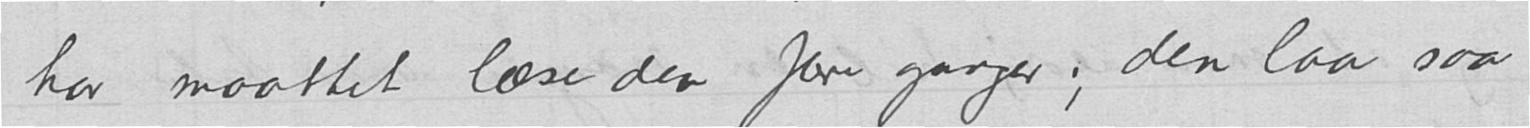har maattet læse den flere ganger; den laa saa
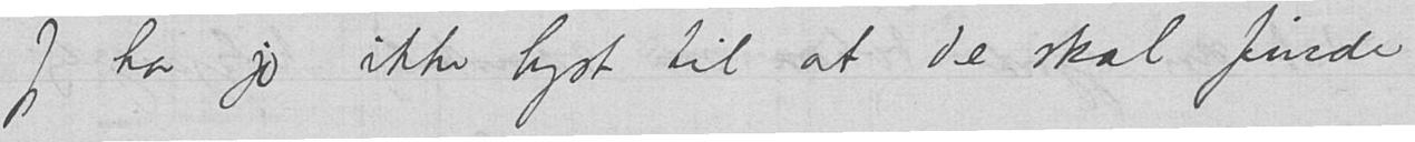Jeg har jo ikke lyst til at De skal finde
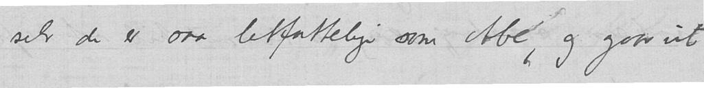selv de er saa letfattelige som Abc, og gaar ut
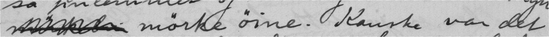mørke øine. Kanske var det
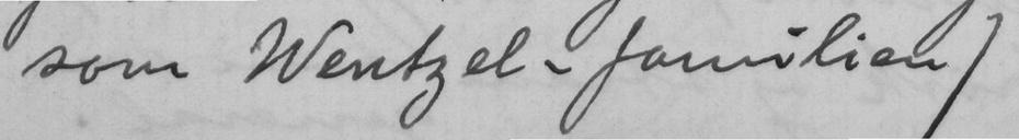som Wentzel-familien)
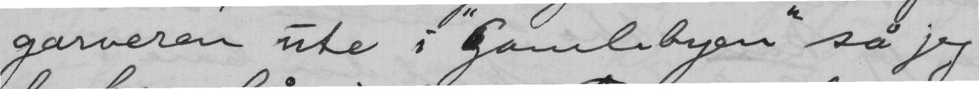garveren ute i "Gamlebyen" så jeg
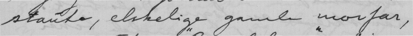staute, elskelige gamle morfar,
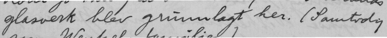glasverk blev grunnlagt her. (Samtidig
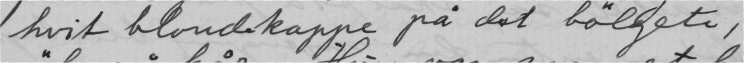hvit blondekappe på det bølgete,
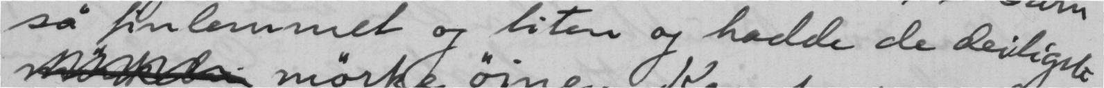så finlemmet og liten og hadde de deiligst
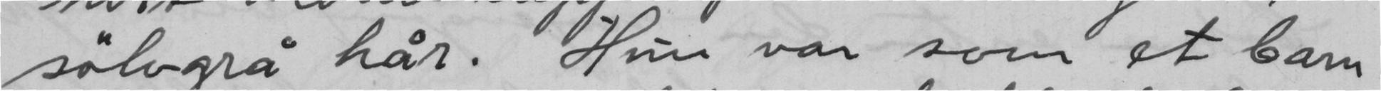sølvgrå hår. Hun var som et barn
